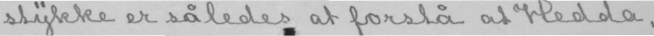stykke er således at forstå at Hedda,
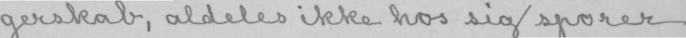gerskab, aldeles ikke hos sig sporer
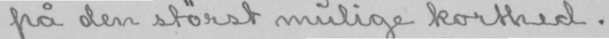på den størst mulige korthed.
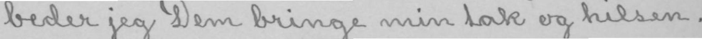beder jeg Dem bringe min tak og hilsen.
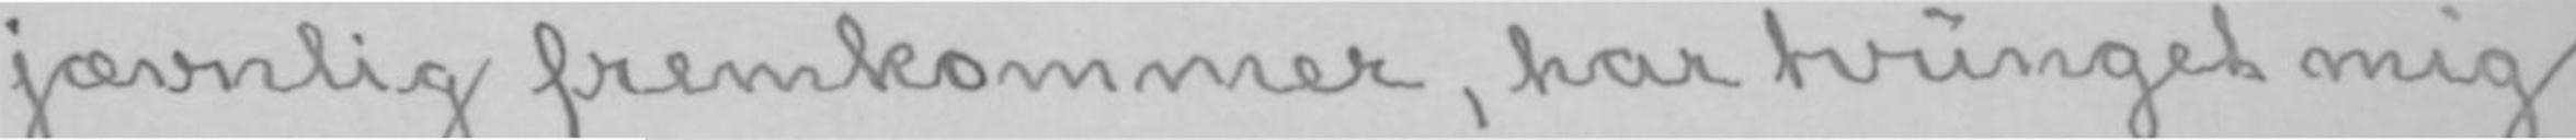jævnlig fremkommer, har tvunget mig
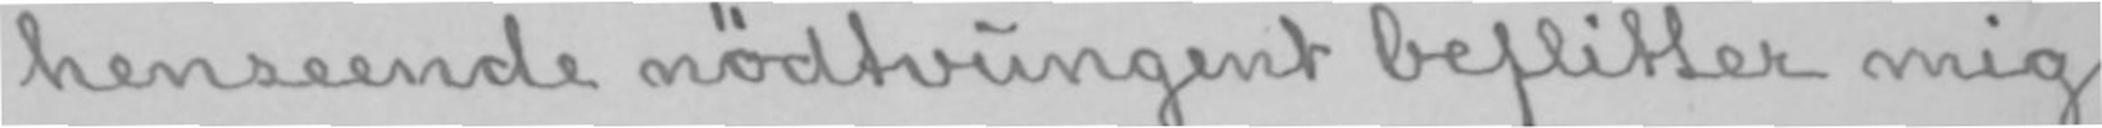henseende nødtvungent beflitter mig
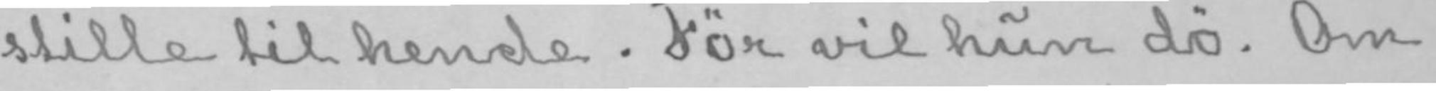stille til hende. Før vil hun dø. Om
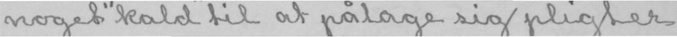noget «kald» til at påtage sig pligter
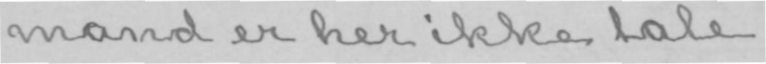mand er her ikke tale.
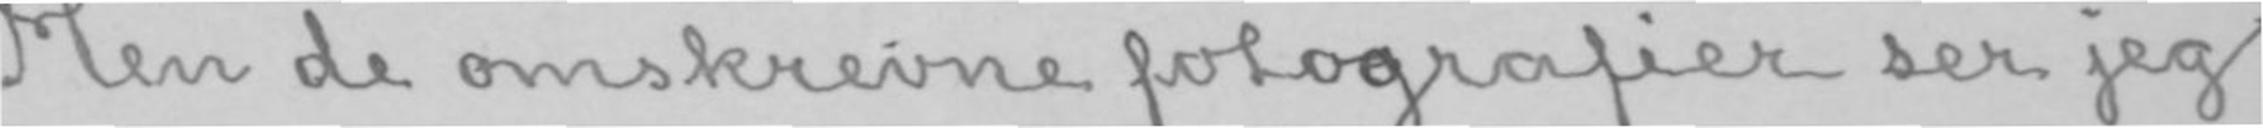Men de omskrevne fotografier ser jeg
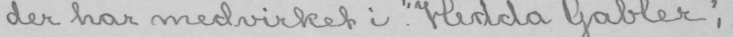der har medvirket i «Hedda Gabler»,
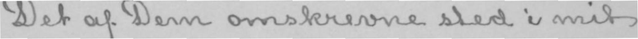Det af Dem omskrevne sted i mit
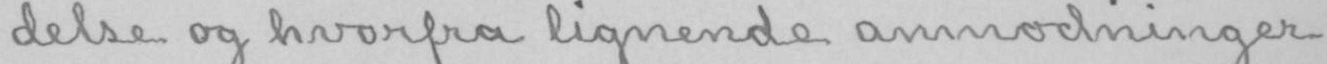delse og hvorfra lignende anmodninger
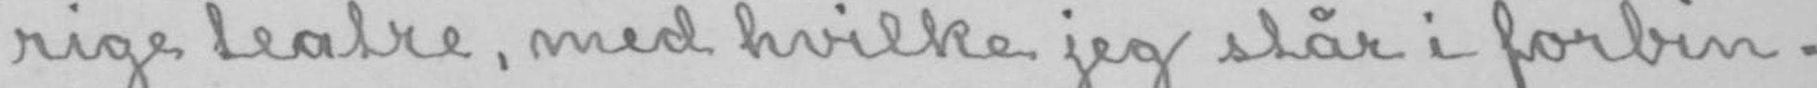rige teatre, med hvilke jeg står i forbin-
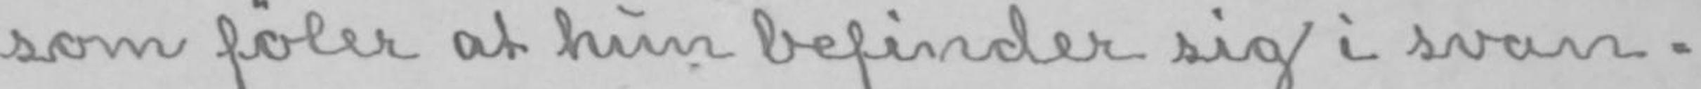som føler at hun befinder sig i svan-
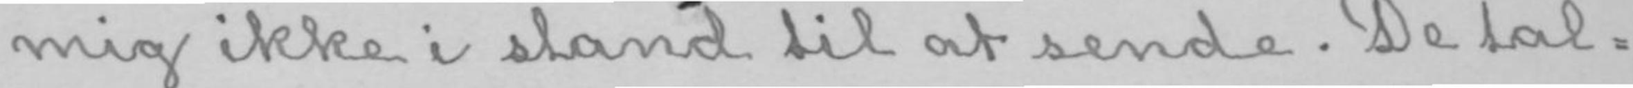mig ikke i stand til at sende. De tal-
